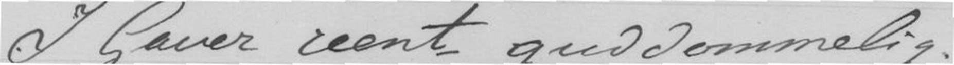I Gaver reent guddommelig.
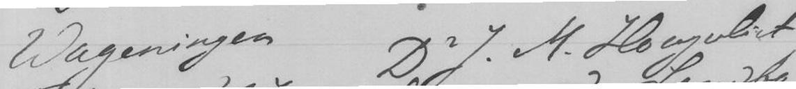Wageningen D J.M.Hoogenlict
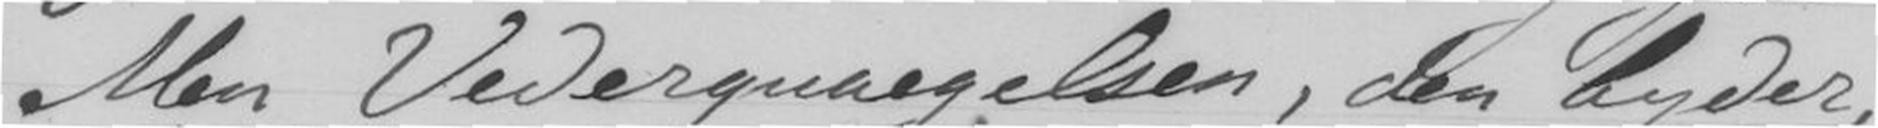Men Vederquaegelsen, den byder,
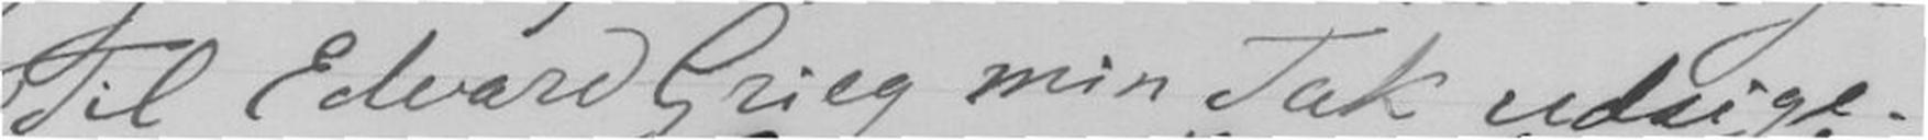til Edvard Grieg min Tak udsige.
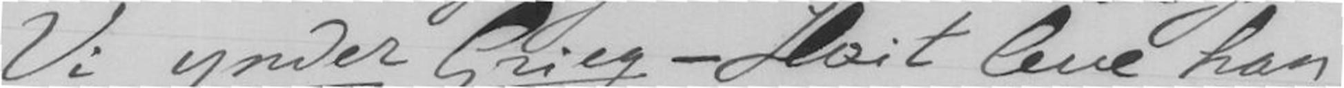Vi ynder Grieg Høit leve han.
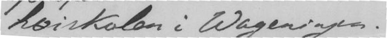høiskolen i Wageningen.
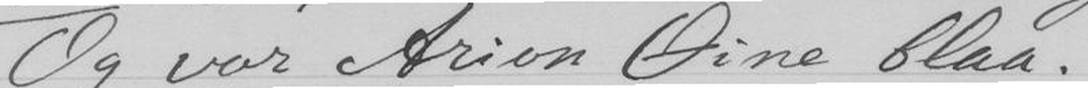Og vor Arion Øine blaa.
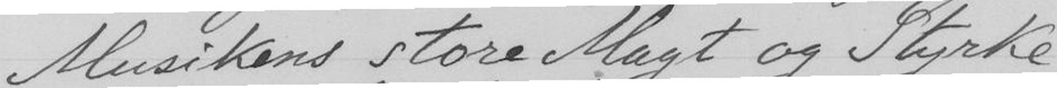Musikens store Magt og Styrke
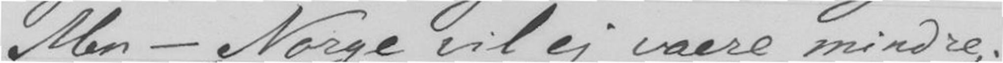Men - Norge vil ej vaere mindre;
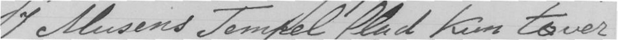I Musens Tempel flad kun tøver
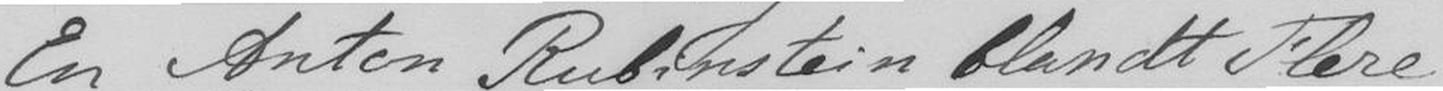En Anton Rubinstein blandt Flere
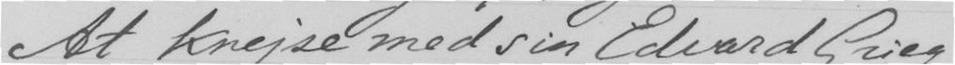At knejse med sin Edvard Grieg
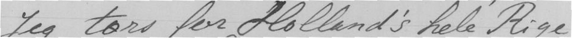Jeg tørs for Holland's hele Rige
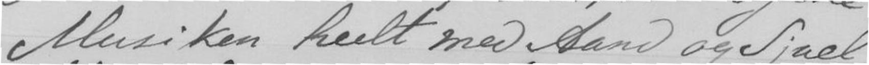Musiken heelt med Aand og Sjael
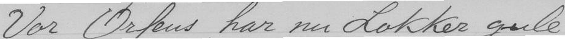Vor Orfeus har nu Lokker gule
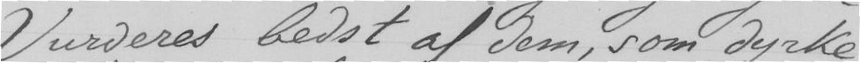Vurderes bedst af dem, som dyrke
